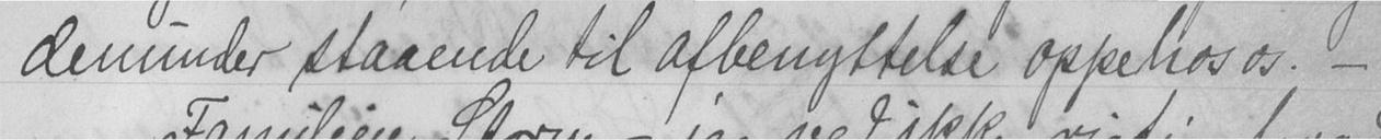denunder staaende til afbenyttelse oppe hos os. -
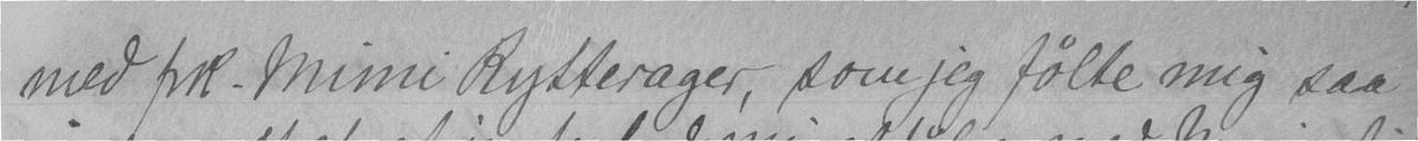med frk. Mimi Rytterager, som jeg følte mig saa
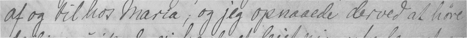af og til hos Maria; og jeg opnaaede derved at høre
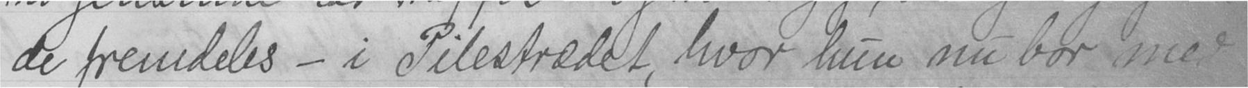de fremdeles - i Pilestrædet, hvor hun nu bor med
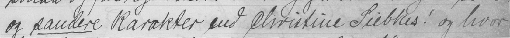og sandere karakter end Christine Siebkes! og hvor
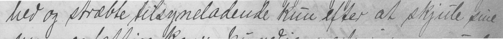hed og stræbte tilsyneladende kun efter at skjule sine
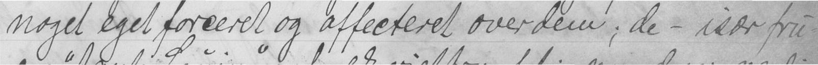noget eget forceret og affecteret over dem; de - især fru-
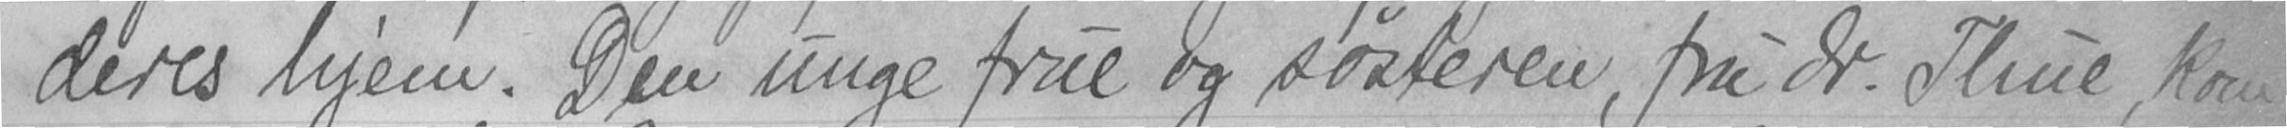deres hjem. Den unge frue og søsteren, fru dr. Thue, kom
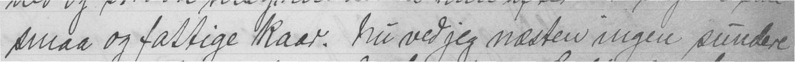smaa og fattige kaar. Nu ved jeg næsten ingen sundere
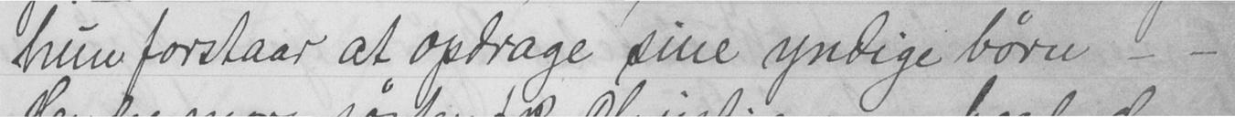hun forstaar at opdrage sine yndige børn - -
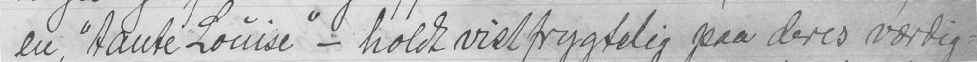en, "tante Louise" - holdt vist frygtelig paa deres værdig-
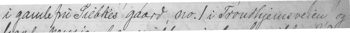i gamle fru Siebkes gaard, no. 1 i Trondhjemsveien, og
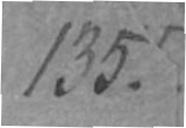135.
